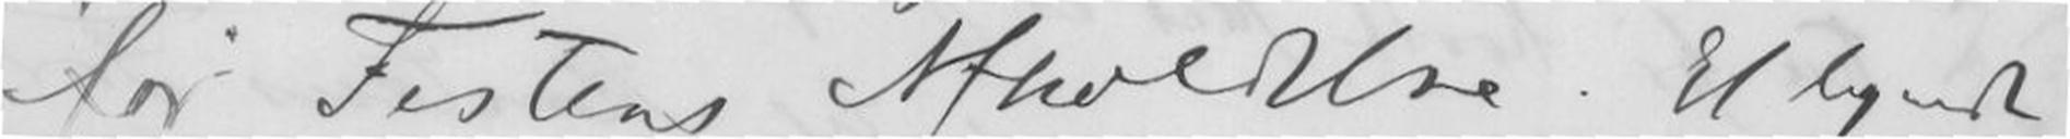før Festen Afholdelse. Et lignende
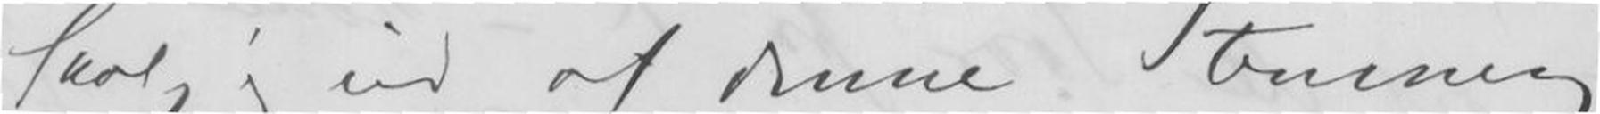Skal jeg ud af denne Stemning
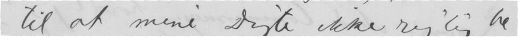jeg til at mine Digte ikke rigtig be-
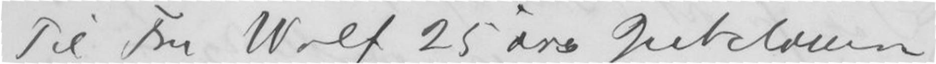Til Fru Wolf 25 års Jubeleum
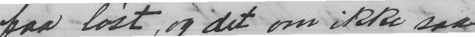faa løst, og det om ikke saa
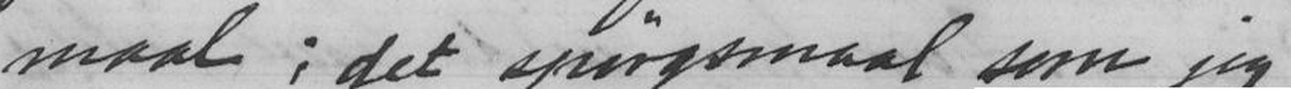maal; det spørgsmaal som jeg
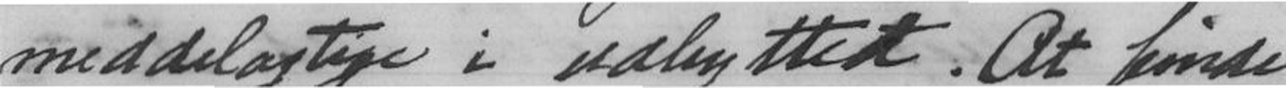meddelagtige i udbyttet. At finde
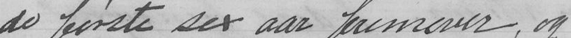de første sex aar fremover, og
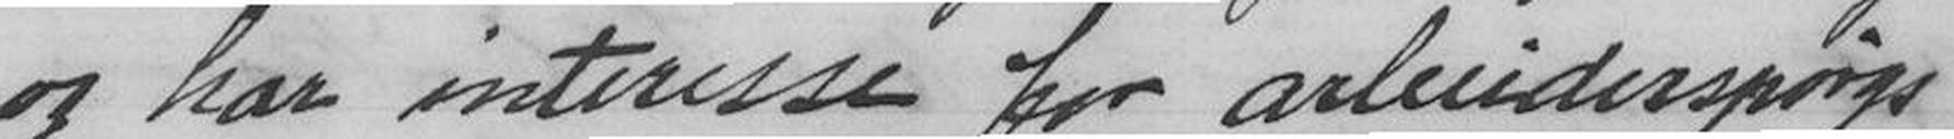og har interesse for arbeiderspørgs
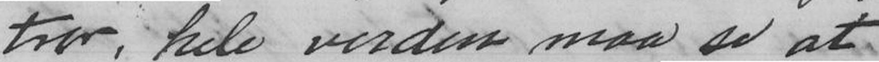tror, hele verden maa se at
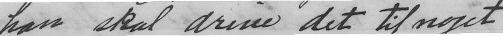han skal drive det til noget.
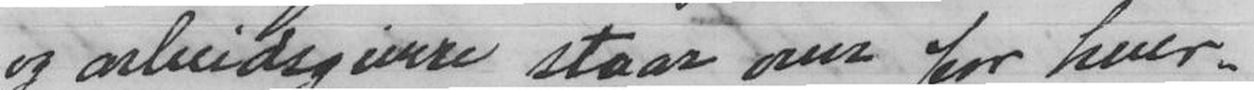og arbeidsgivere staar over for hver-
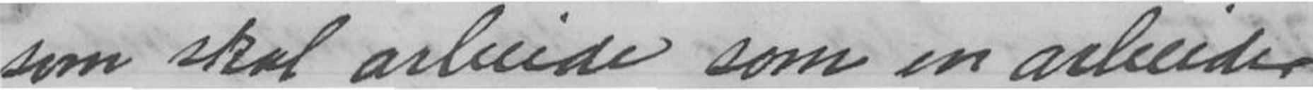som skal arbeide som en arbeider
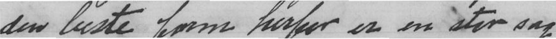den beste form herfor er en stor sag.
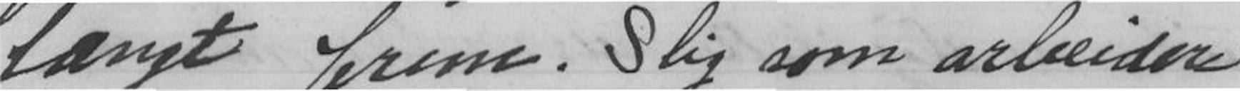langt frem. Slig som arbeidere
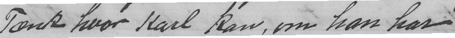Tænk hvor Karl kan, om han har
